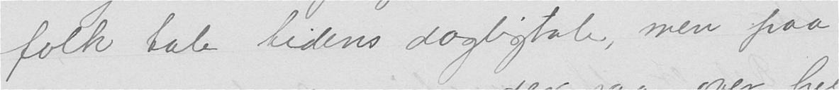folk tale tidens dagligtale, men paa
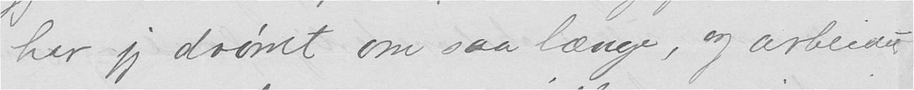har jeg drømt om saa længe, og arbeidet
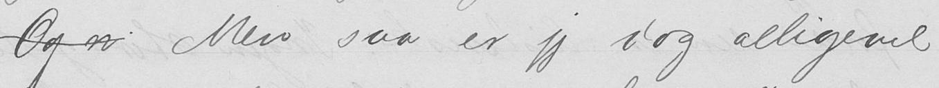Og vi Men saa er jeg dog alligevel
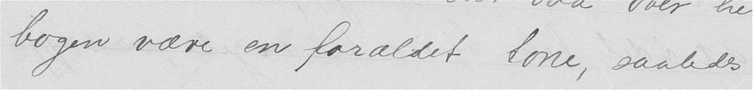bogen være en forældet tone, saaledes
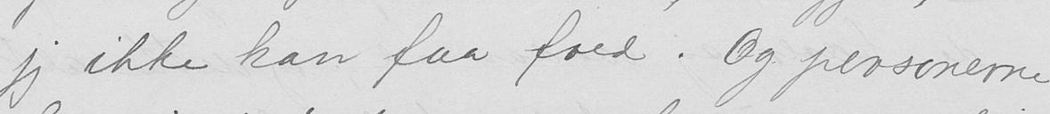jeg ikke kan faa fred. Og personerne
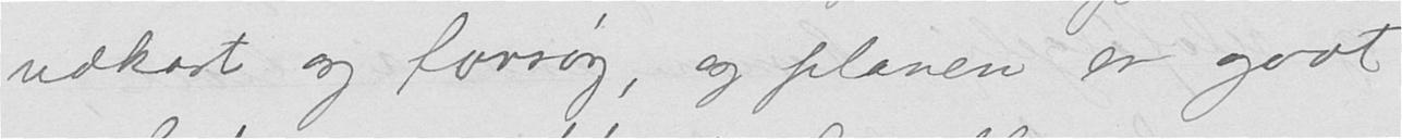udkast og forsøk, og planen er godt
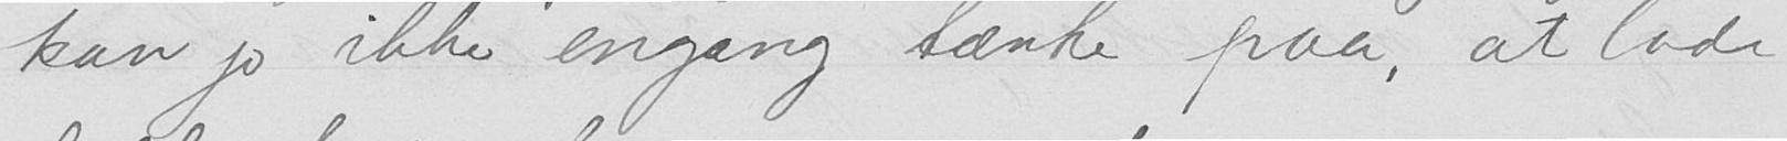kan jo ikke engang tænke paa, at lade
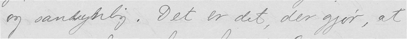og sandsynlig. Det er det, der gjør, at
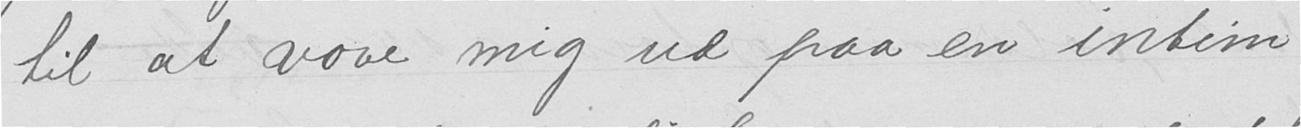til at vove mig ud paa en intim
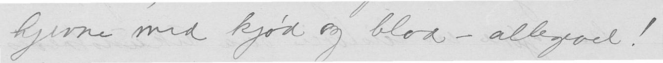hjerne med kjød og blod - alligevel!
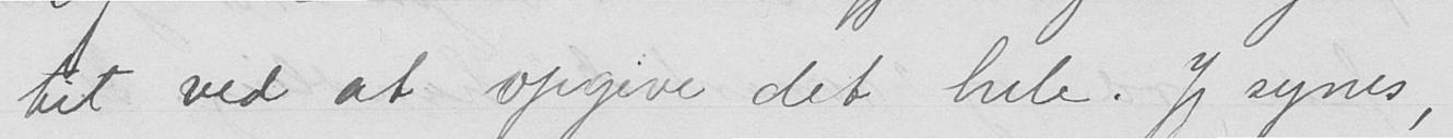tit ved at opgive det hele. Jeg synes,
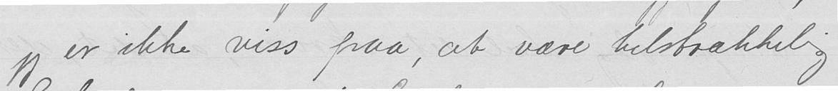jeg er ikke viss paa, at være tilstrækkelig
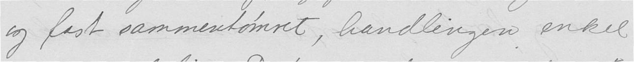og fast sammentømret, handlingen enkel
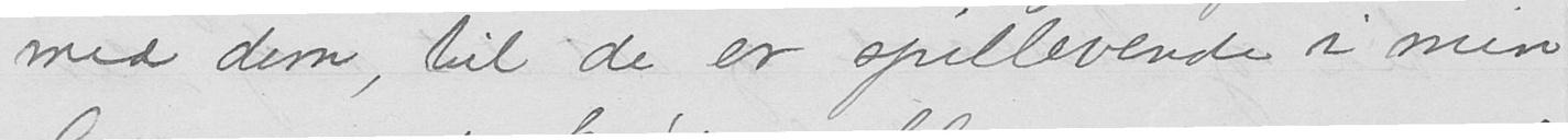med dem, til de er spillevende i min
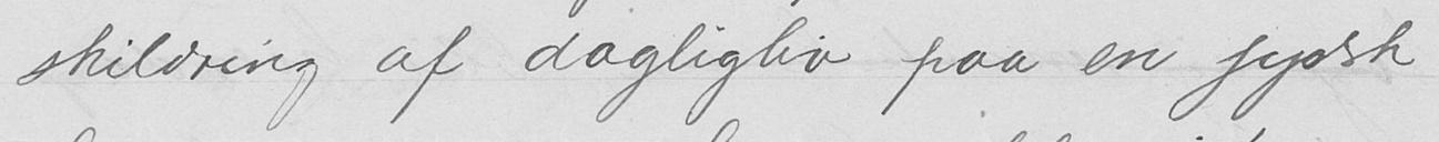skildring af dagligliv paa en jydsk
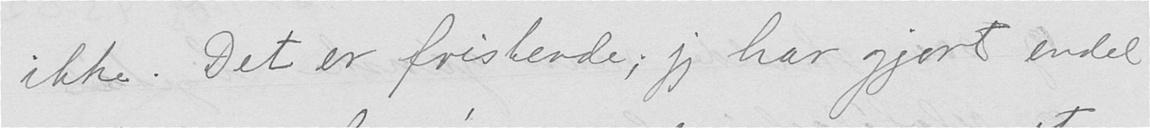ikke. Det er fristende; jeg har gjort endel
